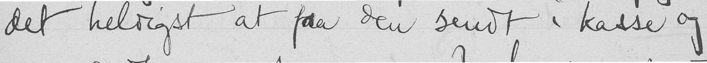det heldigst at faa den sendt i kasse og
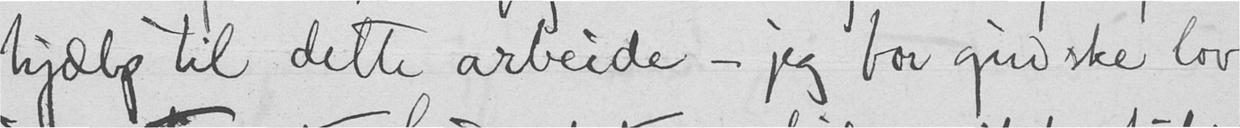hjælp til dette arbeide - jeg bor gud ske lov
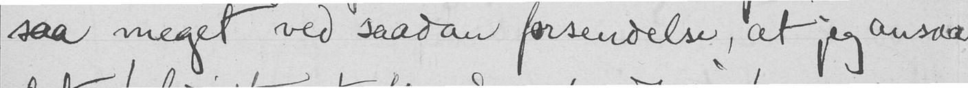saa meget ved saadan forsendelse, at jeg ansaa
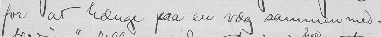for at hænge paa en væg sammen med
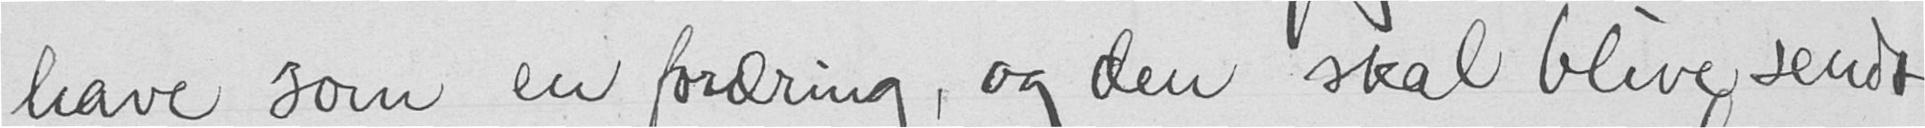have som en fordring, og den skal blive sendt
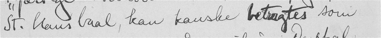St. Hans baal, kan kanske betagtes som
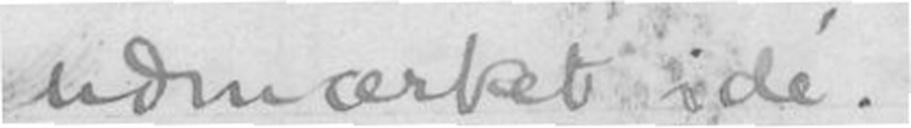udmærket ide.
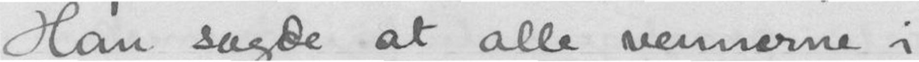Han sagde at alle vennerne i
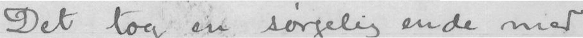Det tog en sørgelig ende med
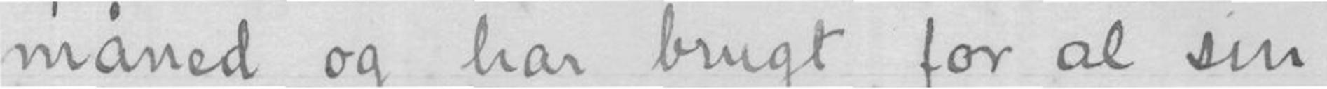måned og har brugt for al sin
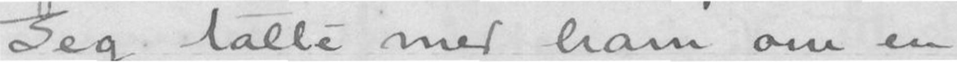Jeg talte med ham om en
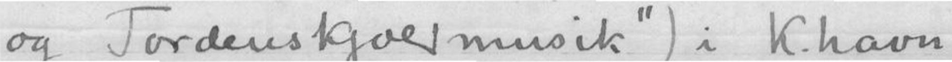og Tordenskjoldmusik) i K.havn
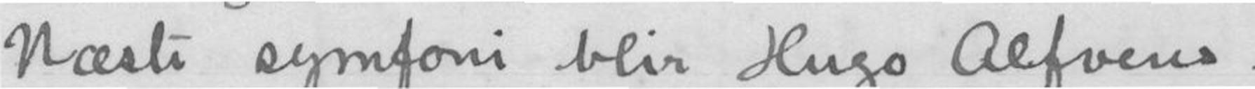Næste symfoni blir Hugo Alfvens.
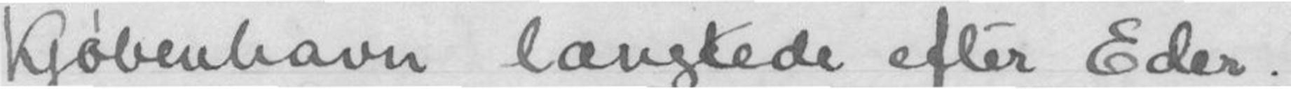København længtede efter Eder.
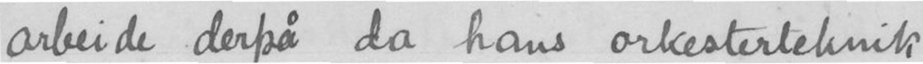arbeide derpå da hans orkesterteknik
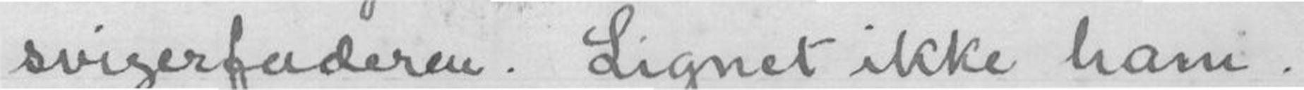svigerfaderen. Lignet ikke ham.
